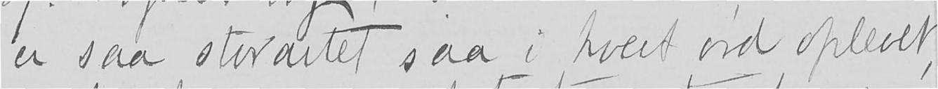er saa storartet saa i hvert ord oplevet,
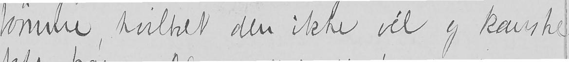tømme, hvilket den ikke vil og kanske
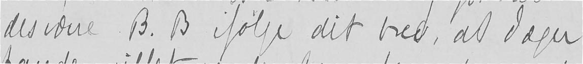desværre B. B. ifølge dit brev, at Jæger
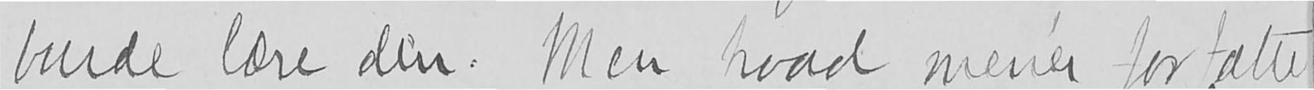burde læse den. Men hvad mener fortatte-
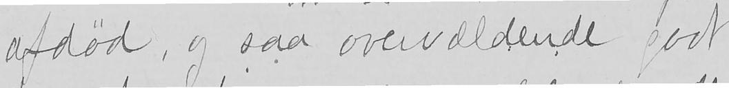afdød, og saa overvældende godt
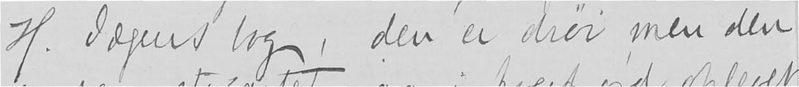H. Jægers bog, den er drøi, men den
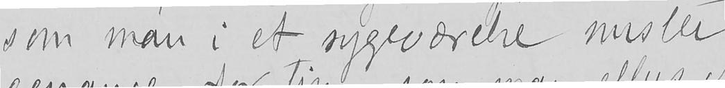som man i et sygeværelse mister
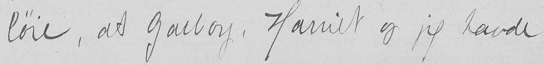løie, at Garborg, Harriet og jeg havde
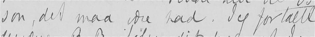son, det maa være had. Jeg fortalte
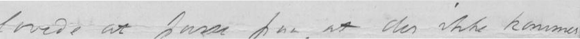lovede at passe paa, at der ikke kommer
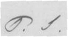P.S.
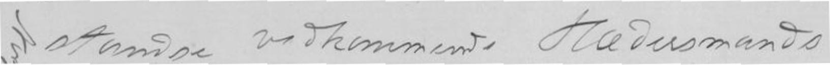standse vedkommende Hædersmands
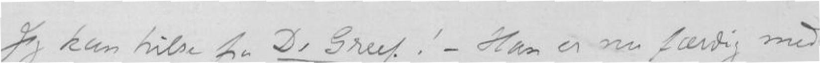Jeg kan hilse fra D. Greef! - Han er nu færdig med
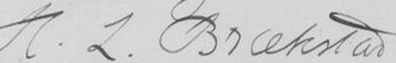H.L. Brækstad
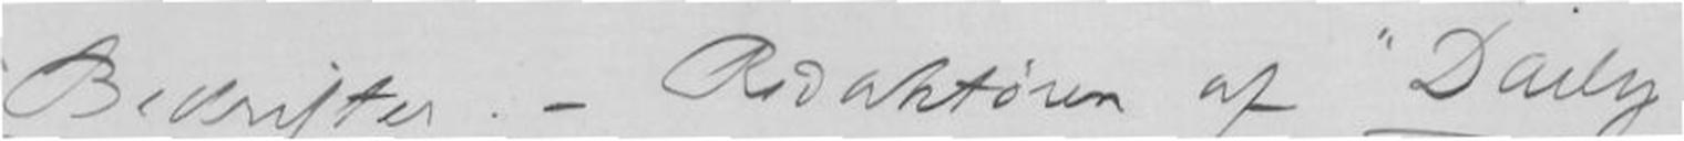Bedrifter. - Redaktøren af "Daily
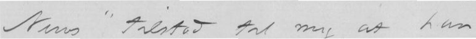News" tilstod til mig, at han
